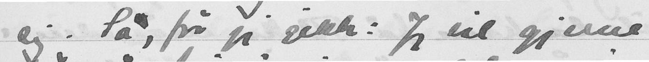sig. Sa, før jeg gikk: Jeg vil gjerne
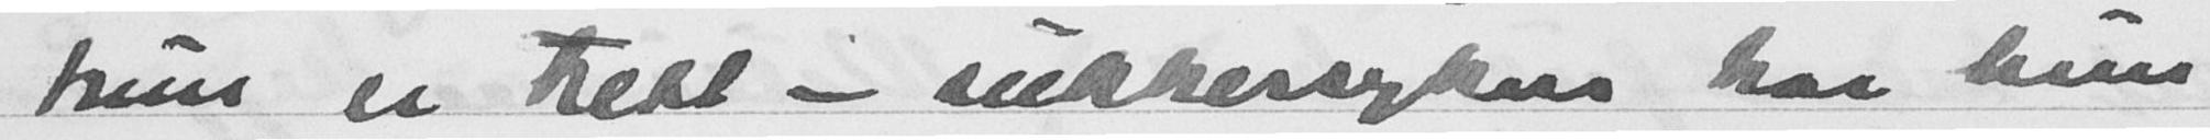hun er trett - sukkersyken har hun
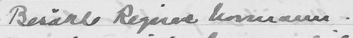Besøkte Regine Normann.
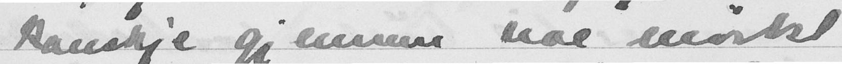kanskje gjennem noe mørkt
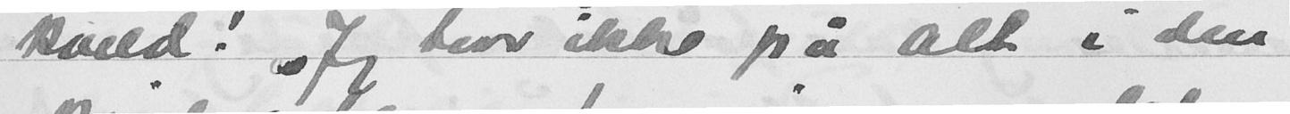kveld! "Jeg tror ikke på Alt i den
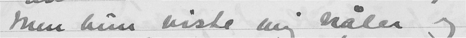Men hun viste mig nåler og
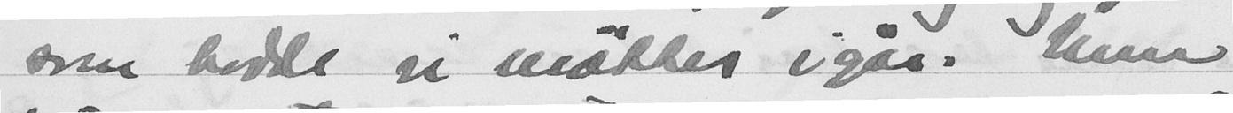som hadde vi møttes igår. Men
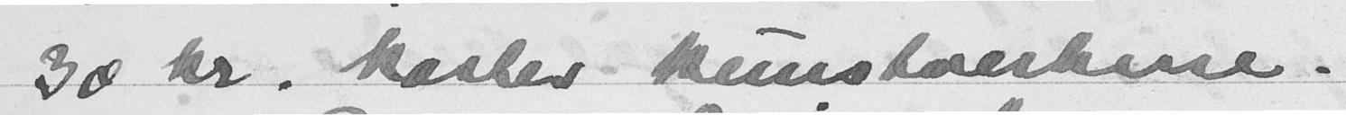30 kr. koster kunstverkene.
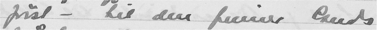først - til den finner Guds
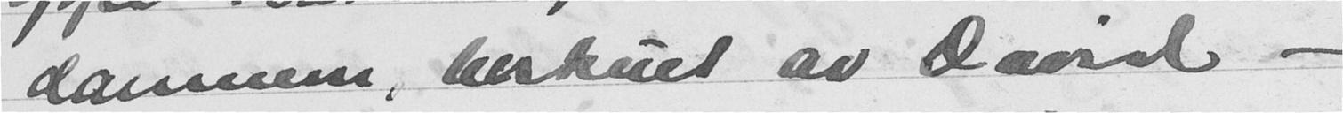dammen, beskuet av David -
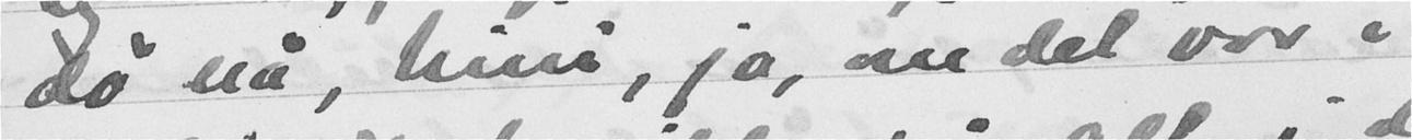dø nu, Nini, ja, om det var i
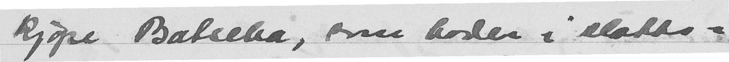kjøpe Batseba, som bader i slotts-
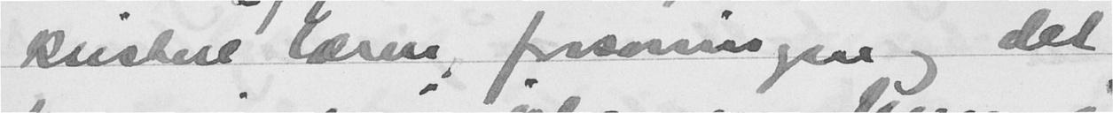kristne læren, forsoningne og det
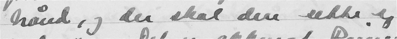hånd, og der skal den sette sig
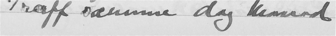Traff samme dag Monrad
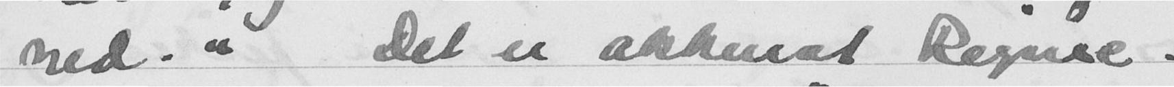ned." Det er akkurat Regine.
